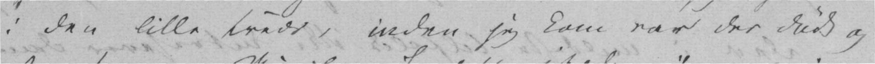i den lille Kreds, inden jeg kom var der dødt og
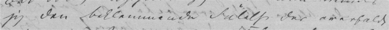jeg den beklemmende Følelse der overfaldt
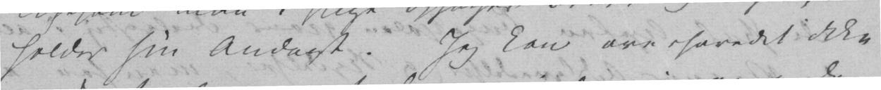holder sin Andagt. Jeg kan overhovedet ikke
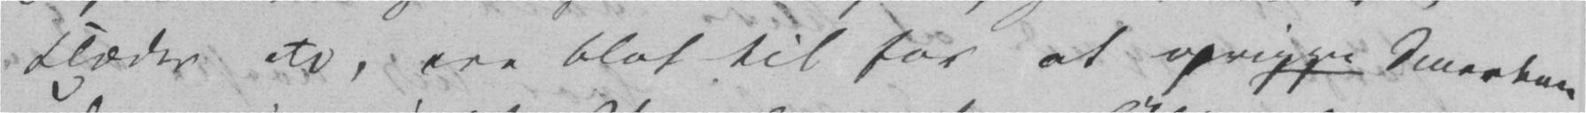Klæder etc, ere blot til for at oprippe Smerten
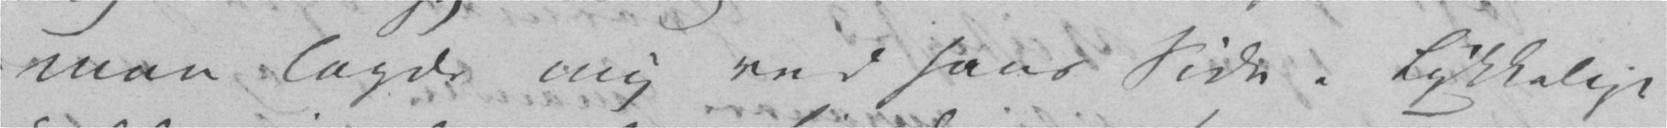man lagde mig ved hans Side. Lykkelige
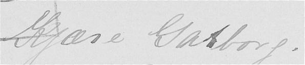Kjære Garborg!
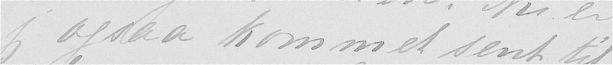jeg ogsaa kommet sent til
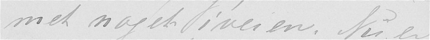met noget i veien. Nu er
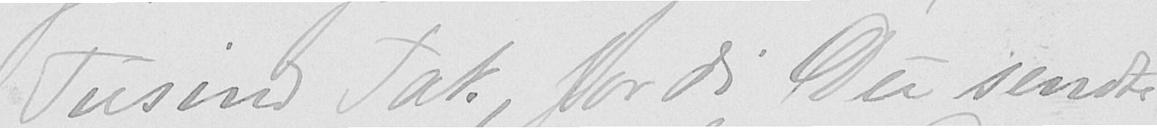Tusind Tak fordi Du sendte
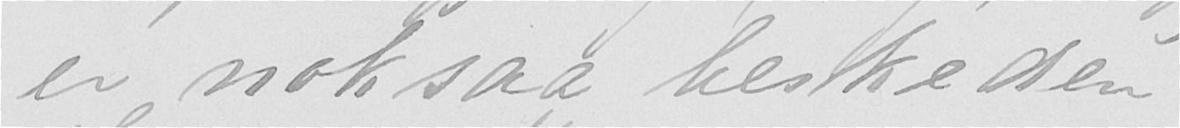er noksaa beskeden.
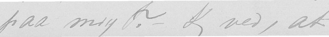paa mig? Jeg ved, at
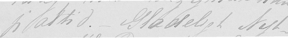jeg altid. Glædeligt Nyt-
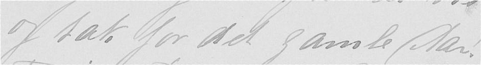og tak for det gamle Aar!
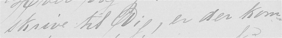skrive til Dig, er der kom-
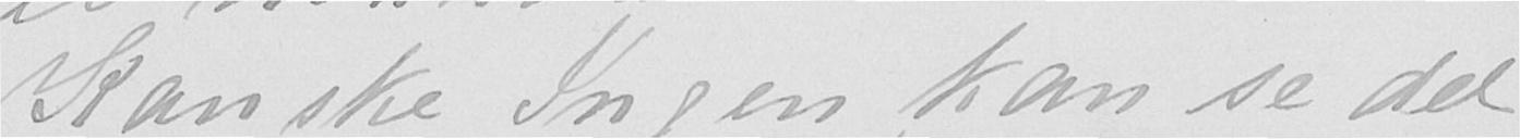Kanske Ingen kan se det
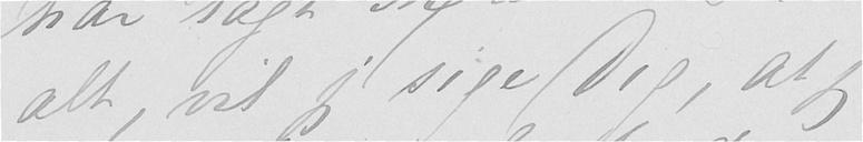alt, vil jeg sige Dig, at jeg
